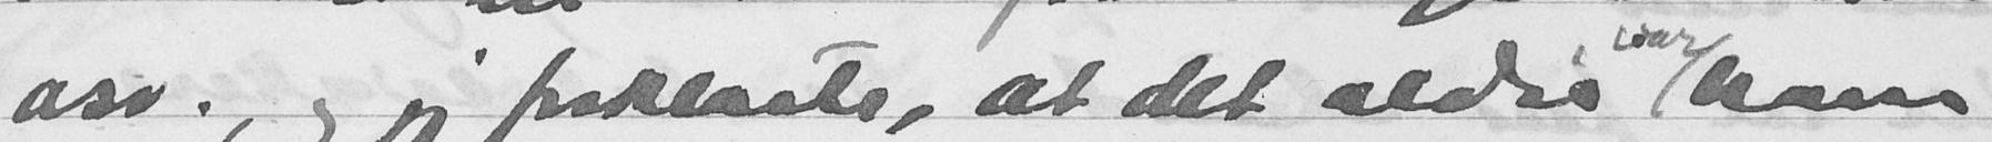osv., og jeg forklarte, at det aldri var ham
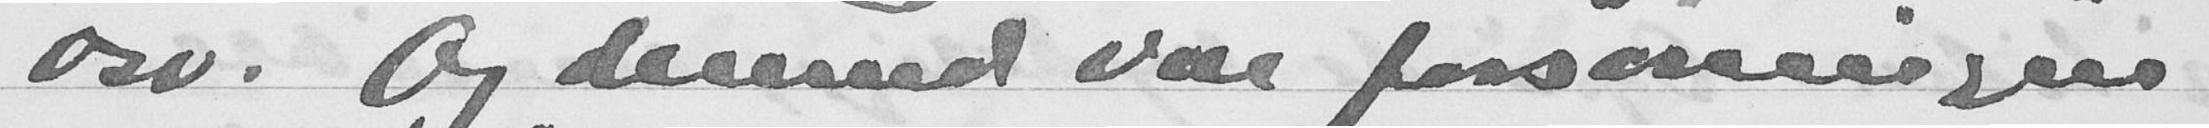Osv. Og dermed var forsoningen
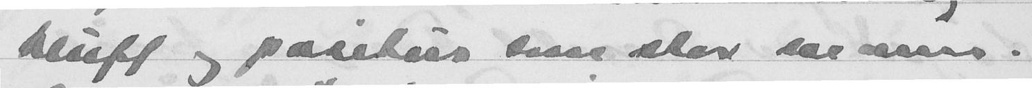bluff og positur som stor mann.
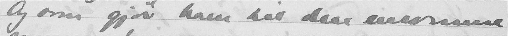Og som gjør ham til den ensomme
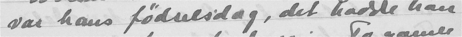var hans fødselsdag, det hadde han
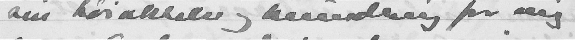sin høiaktelse og beundring for mig
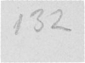132
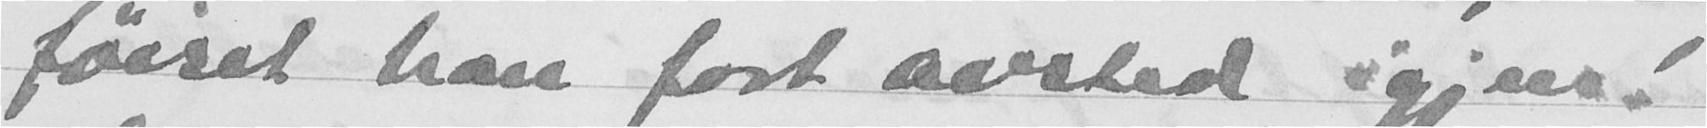føiset han fort avsted igjen!
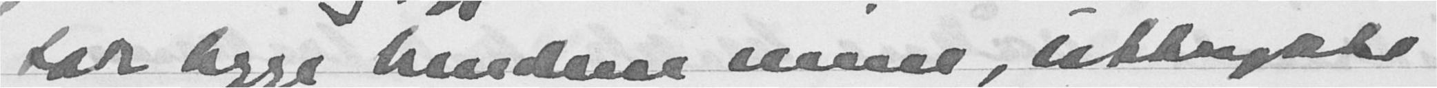tok begge hendene mine, uttrykte
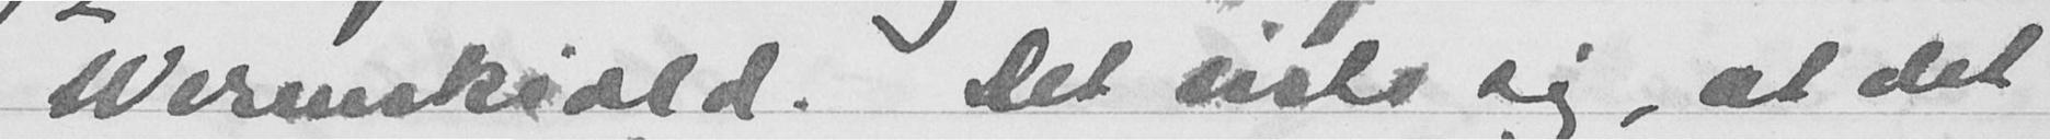Werenskiold. Det viste sig, at det
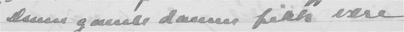Denne gamle damen fikk være
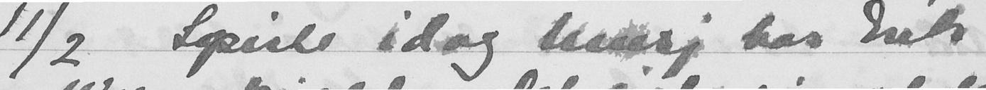11/2 Spiste idag lunsj hos Erik
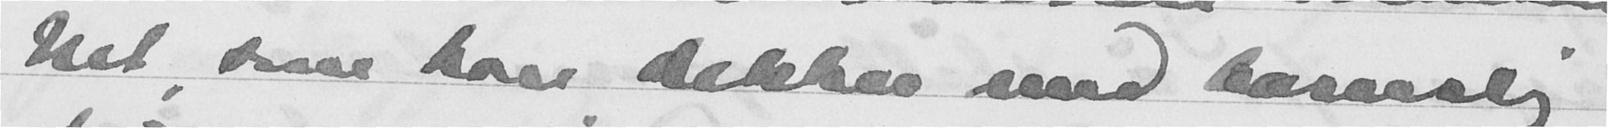het som han dekker med barnslig
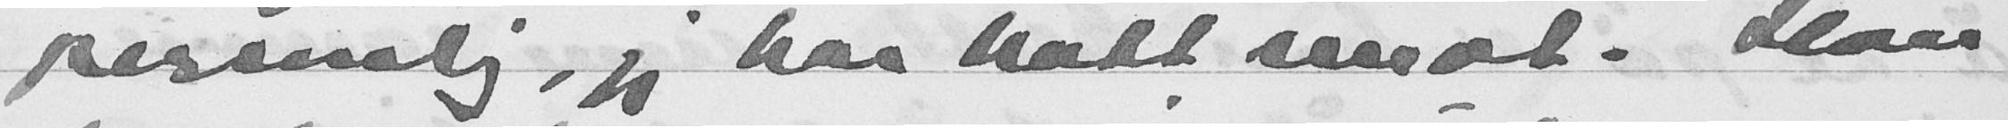personlig, jeg har hatt imot. Han
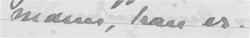mann, han er.
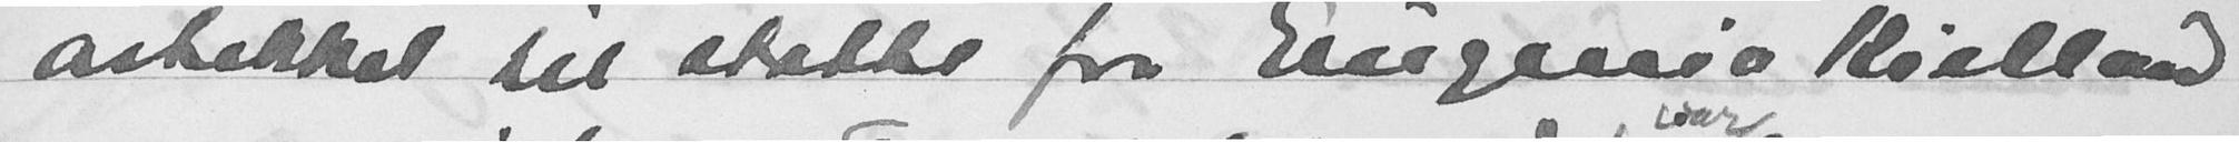artikkel til støtte for Eugenia Kielland
